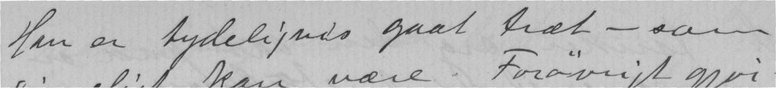Han er tydeligvis gaat træt - som
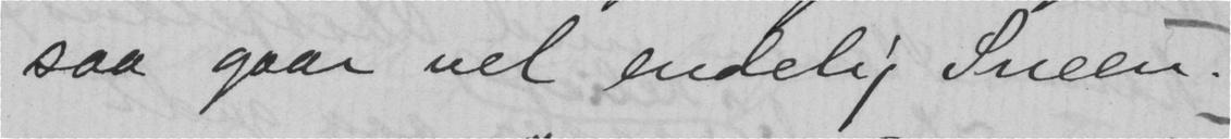saa gaar vel endelig Sneen.
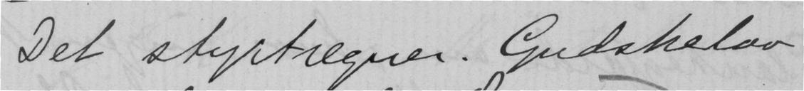Det styrtregner. Gudskelav
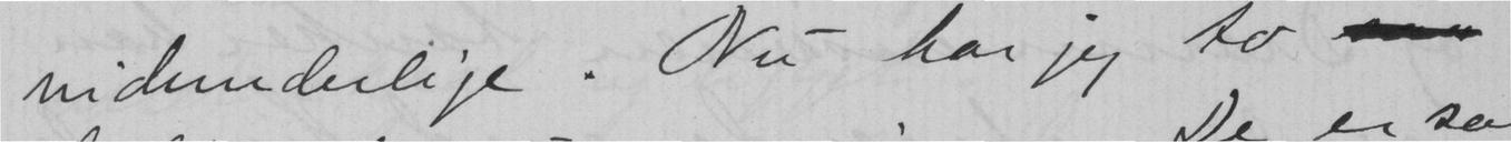vidunderlige. Nu har jeg to
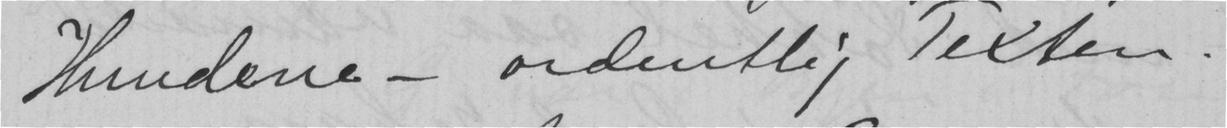Hundene - ordentlig Texten.
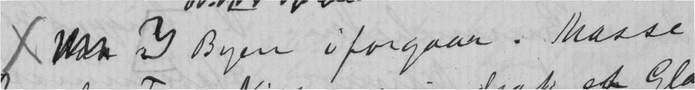x  I Byen iforgaar. Masse
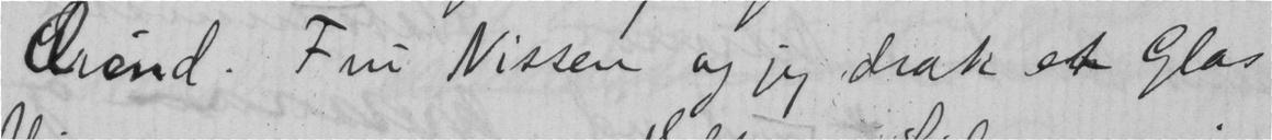Ærend. Fru Nissen og jeg drak et Glas
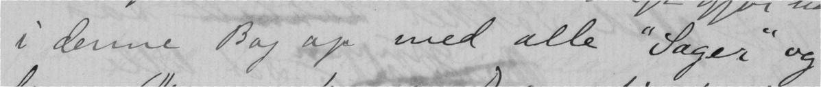i denne Bog op med alle "Sager" og
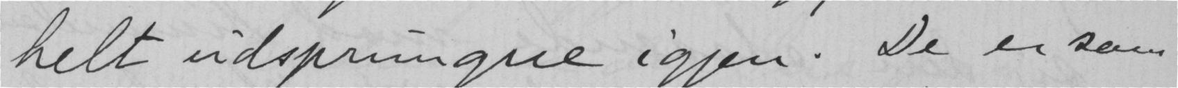helt udsprungne igjen. De er som
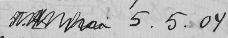5.5.04
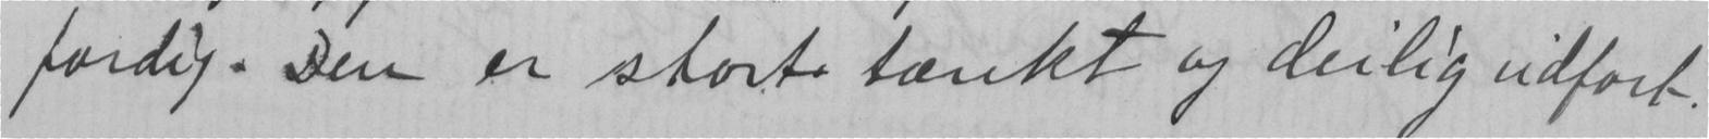færdig. Den er stort tænkt og deilig udført.
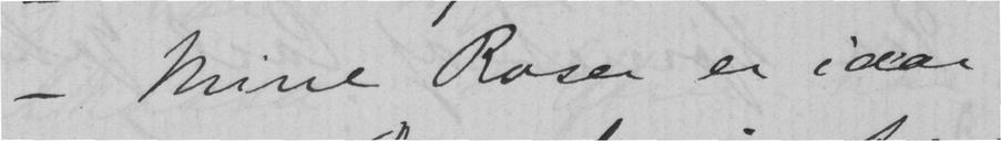- Mine Roser er iaar
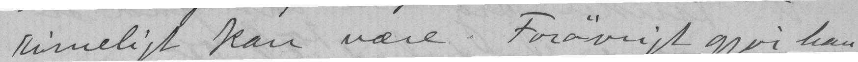rimeligt kan være. Forøvrigt gjør han
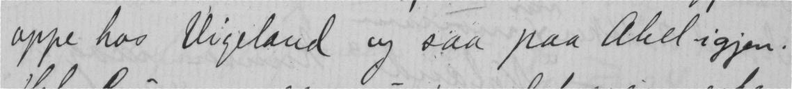oppe hos Vigeland og saa paa Abel igjen.
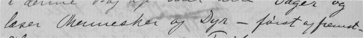læser mennesker og Dyr - først og fremst -
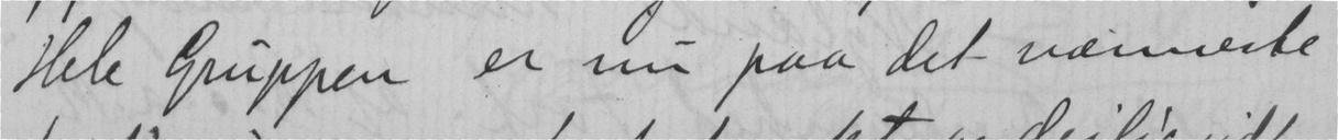Hele Gruppen er nu paa det nærmeste
